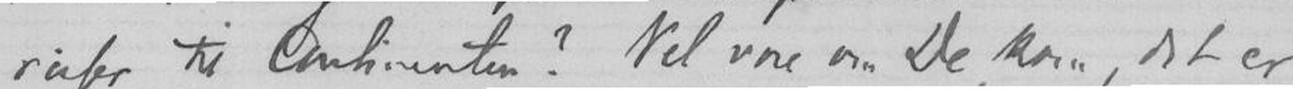reiser til Continenten? Vel vare om De kan", det er
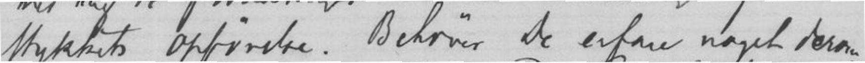stykkets opførelse. Behøver De erfare noget derom
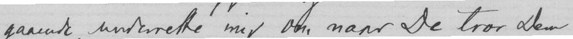gaande underrette mig om naar De tror Dem
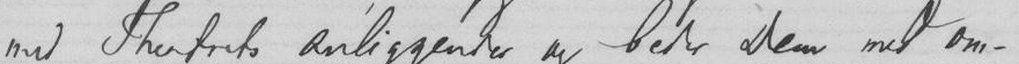med Theatrets anliggende og beder Dem med om-
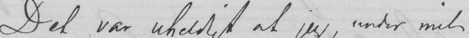Det var uheldigt at jeg, under mit
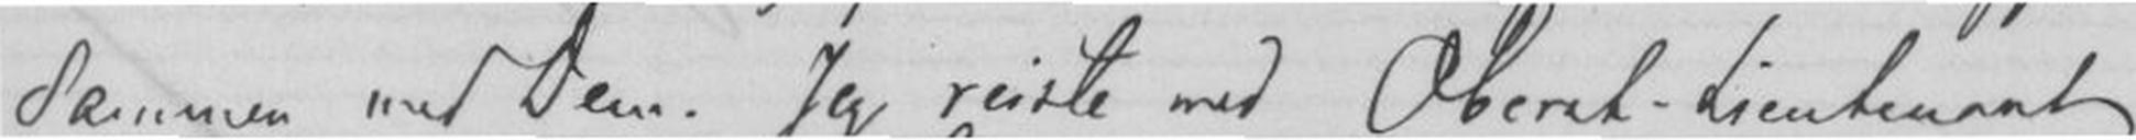Sammen med dem. Jeg reiste med Oberst-Lieutenant
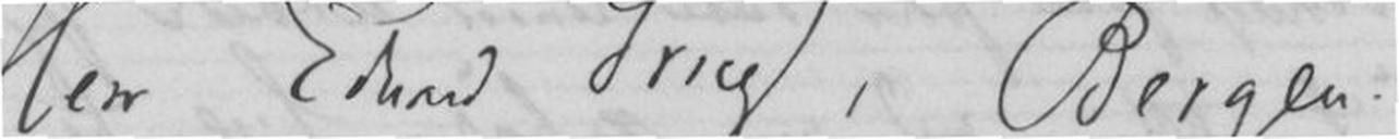Herr Edvard Grieg, Bergen.
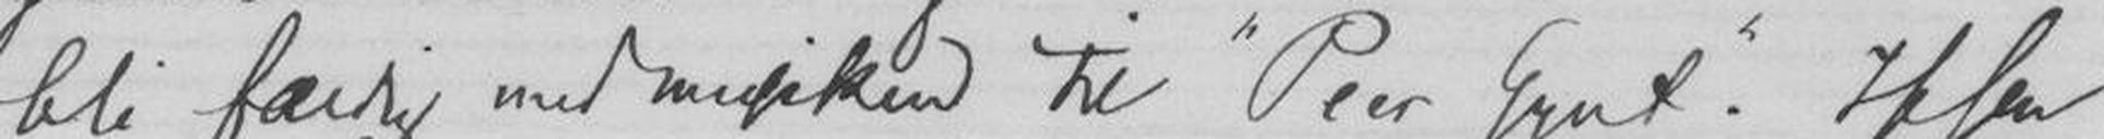bli færdig med musiken til "Peer Gynt". Ibsen
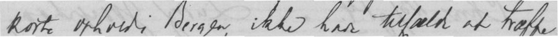korte ophold i Bergen, ikke har tilfælde at træffe
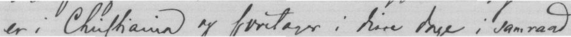er i Christiania og foretager i disse dage i samraad
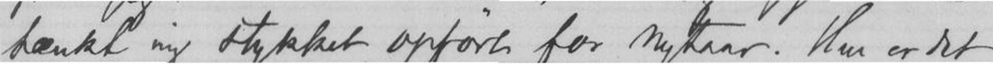tænkt mig stykket opført for nytaar. Her er det,
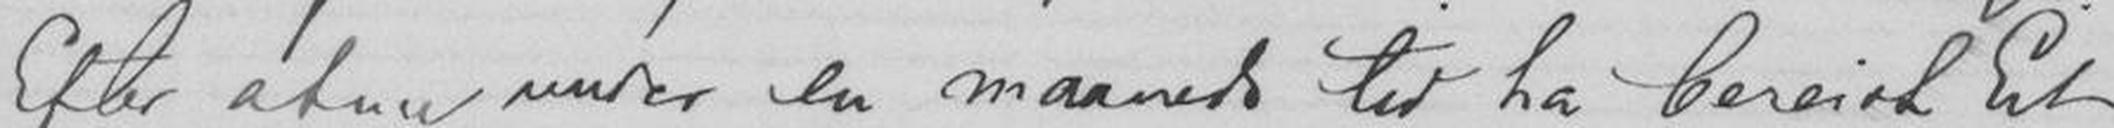Efter at nu under en maaneds tid ha bereist Et
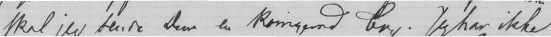skal jeg sende Dem en korrigerd bog. Jeg har ikke
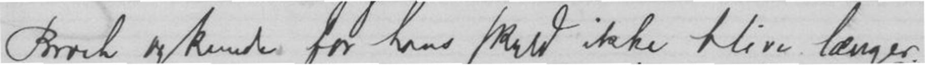Broch og kunde for hans skuld ikke blive længer.
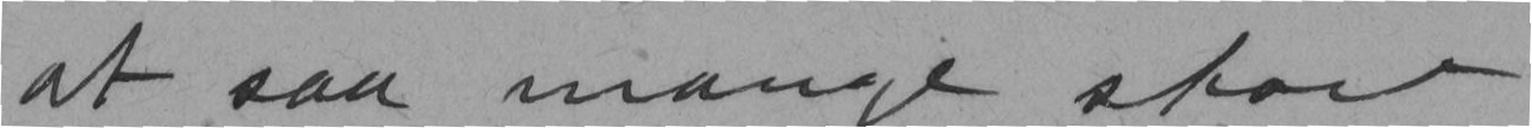at saa mange store??
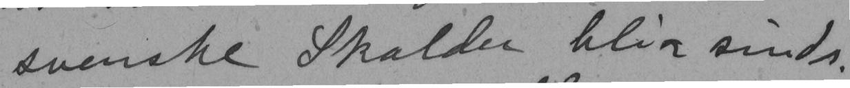svenske Skalder blir sinds.
